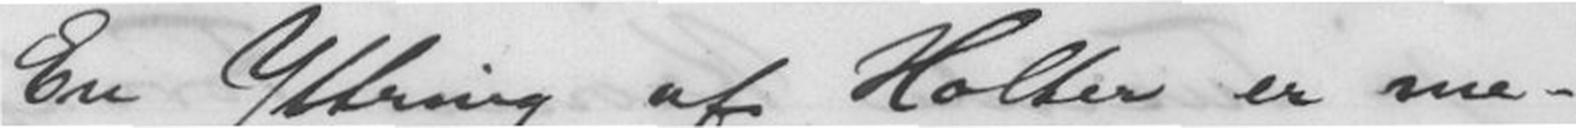En Yttring af Holter er me-
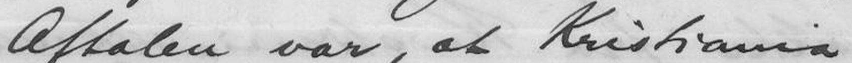Aftalen var, at Kristiania
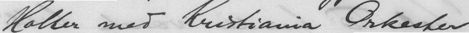Holter med Kristiania Orkester
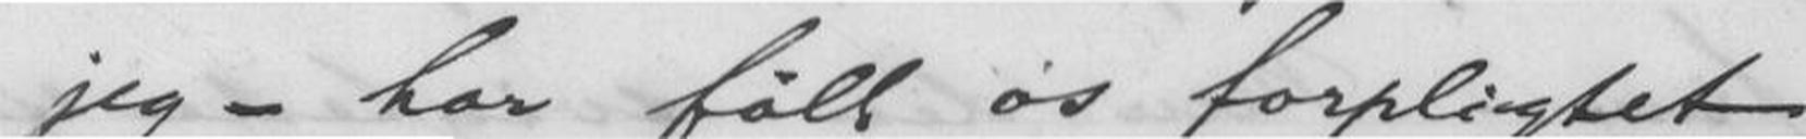jeg - har følt os forpligtet
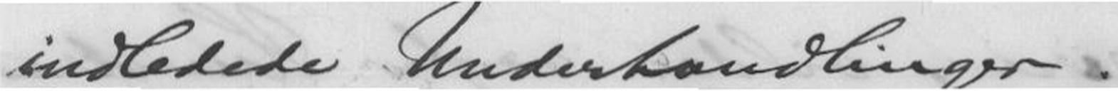indledede Underhandlinger.
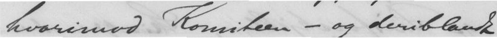hvorimod Komiteen - og deriblandt
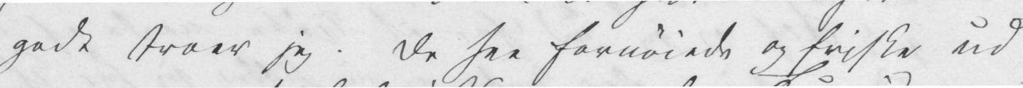godt troer jeg. De see fornøiede og friske ud
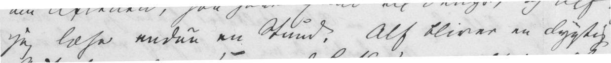jeg læse endnu en Stund. Alf bliver en dygtig
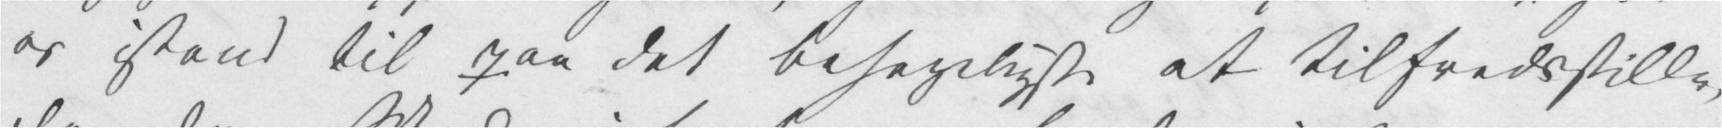os istand til paa det behageligste at tilfredsstille,
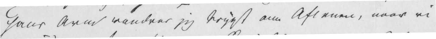hans Arm vandrer jeg trygt om Aftenen, naar vi
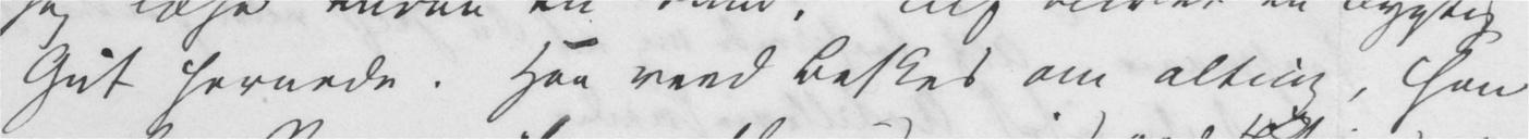Gut hernede. Han veed Besked om alting, han
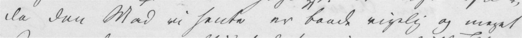da den Mad vi hente er baade rigelig og meget
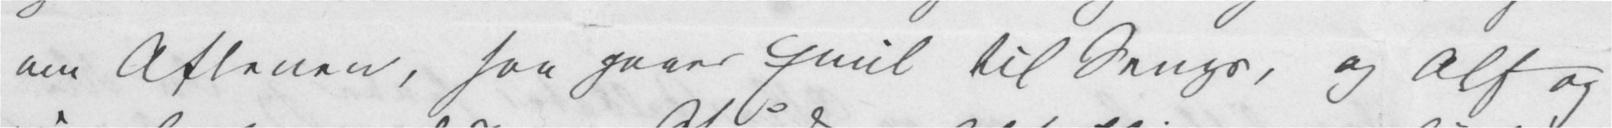om Aftenen, saa gaaer Emil til Sengs, og Alf og
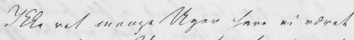Ikke ret mange Uger have vi været
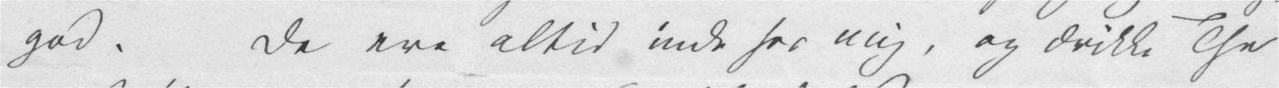god. De ere altid inde hos mig, og drikke The
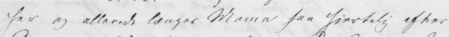her og allerede længes Mama saa hjertelig efter
